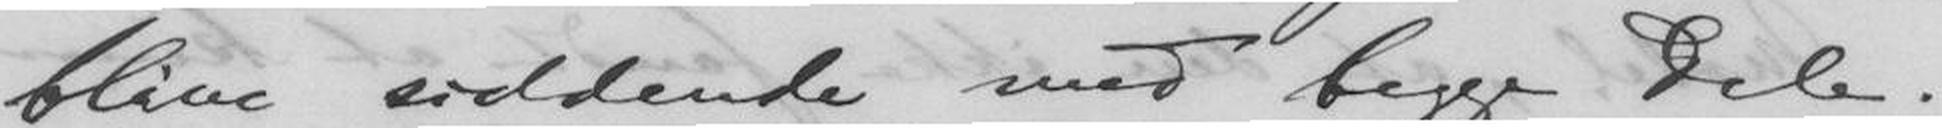blive siddende med begge Dele.
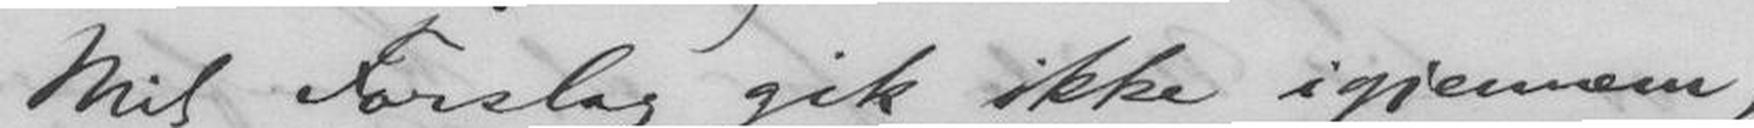Mit Forslag gik ikke gjennem,
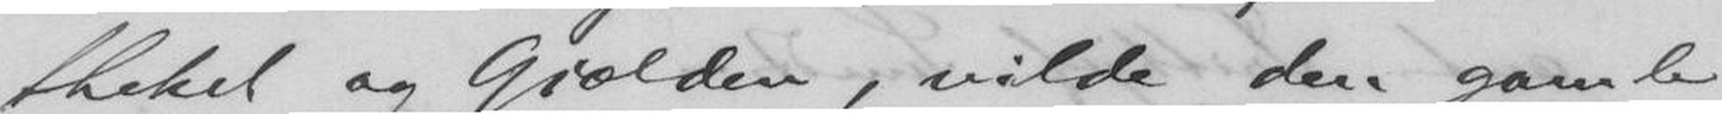theket og Gjælden, vilde den gamle
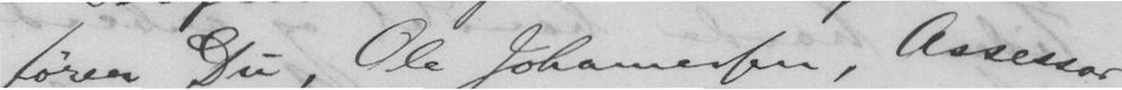tører Du, Ole Johannessen, Assessor
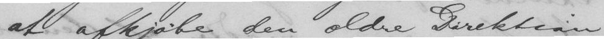at afkjøbe den ældre Direktion
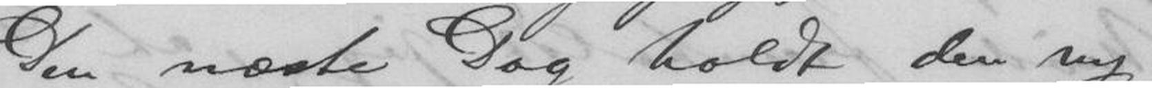Den næste Dag holdt den ny-
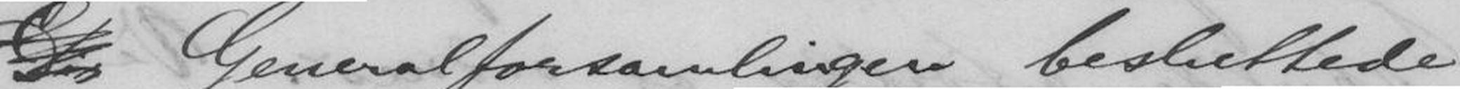Generalforsamlingen besluttede
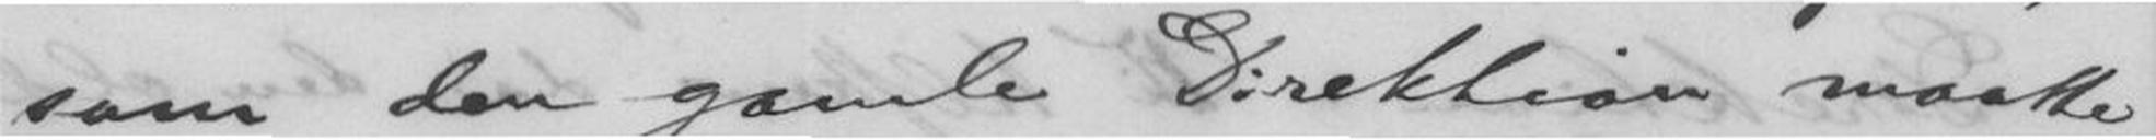som den gamle Direktion maatte
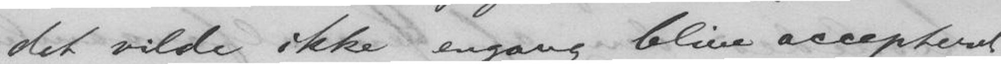det vilde ikke engang blive accepteret
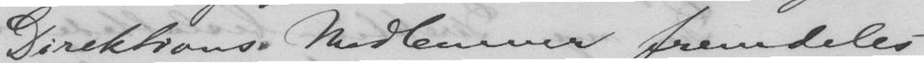Direktions Medlemmer fremdeles
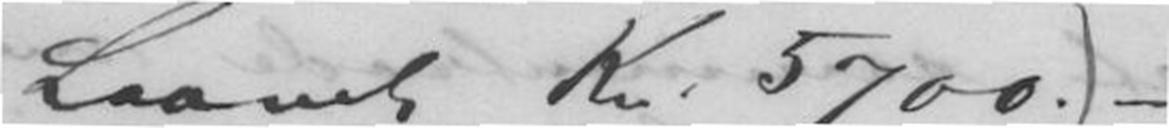Laanet Kr 5700.) -
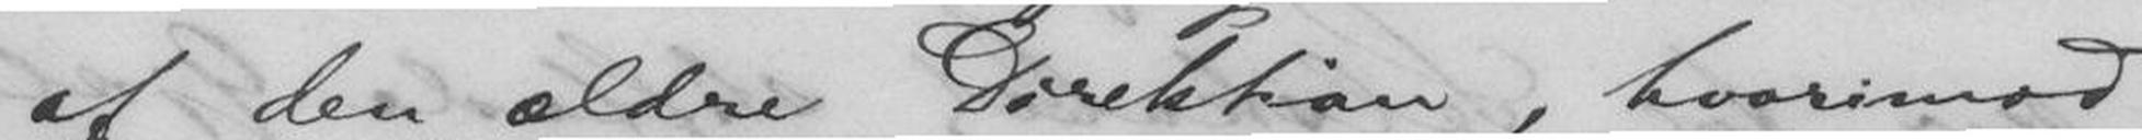af den ældre Direktion, hvorimod
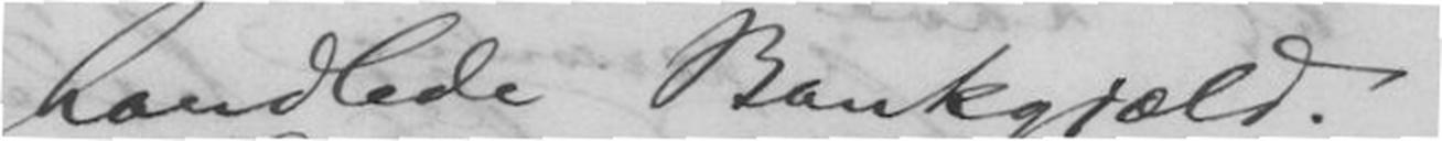handlede Bankgjæld.
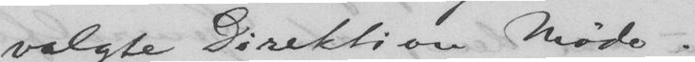valgte Direktion Møde.
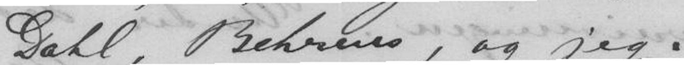Dahl, Behrens, og jeg.
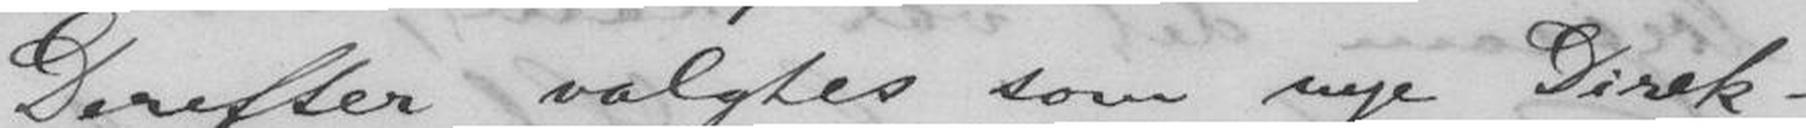Derefter valgtes som nye Direk-
